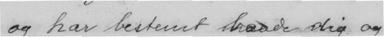og har bestemt baade dig og
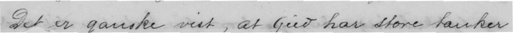Det er ganske vist, at Gud har store tanker
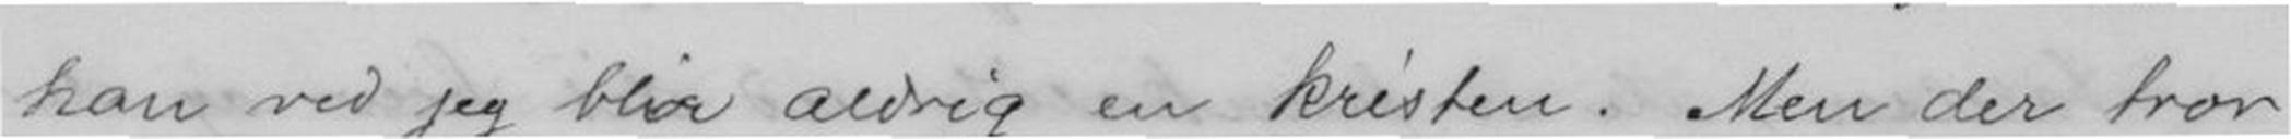han ved jeg blir aldrig en kristen. Men der tror
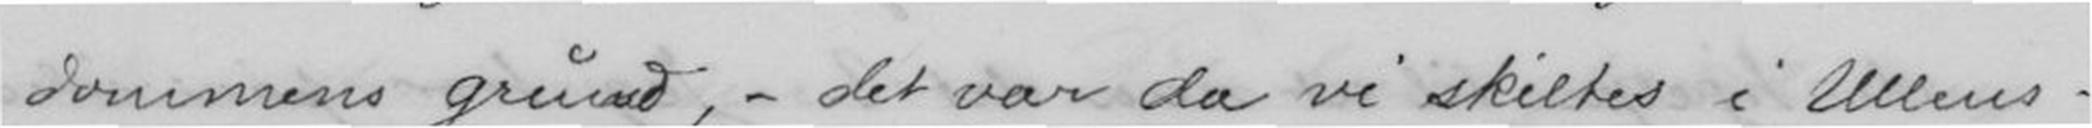dommens grund, - det var da vi skiltes i Ullens -
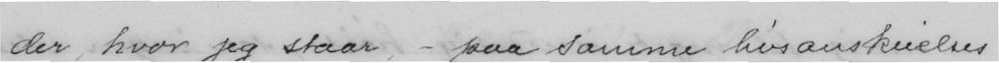der hvor jeg staar, - paa samme livsanskuelses
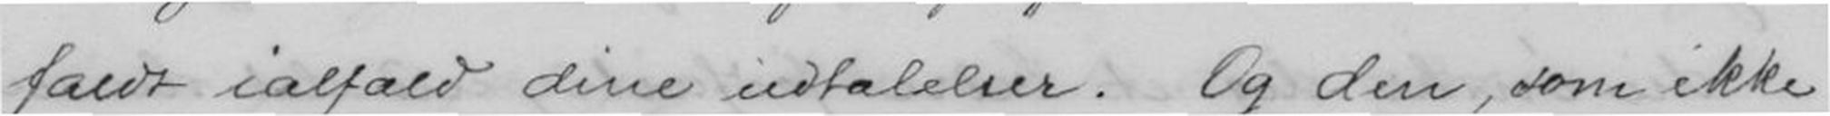faldt ialfald dine udtalelser. Og den, som ikke
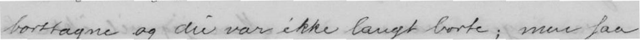borttagne og du var ikke langt borte; men saa
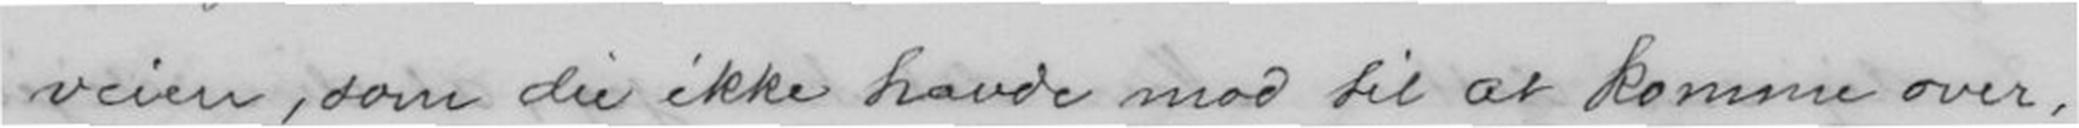veien, som du ikke havde mod til at komme over,
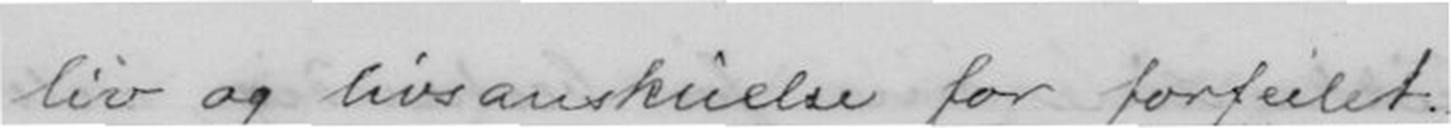liv og livsanskuelse for forfeilet.
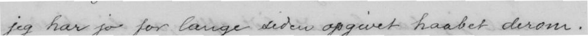jeg har jo for længe siden opgivet haaabet derom.
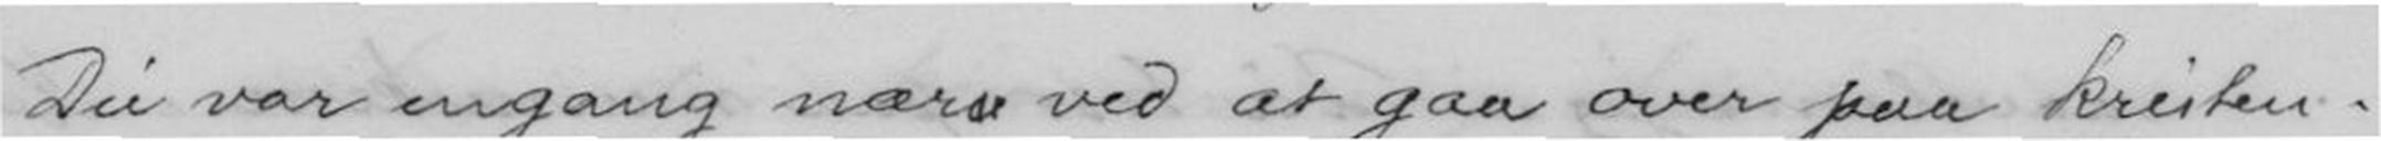Du var engang nære ved at gaa over paa kristen -
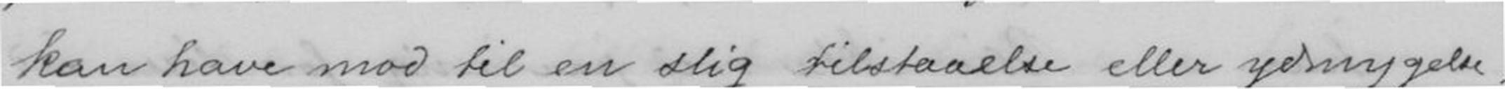kan have mod til en slig tilstaaelse eller ydmygelse,
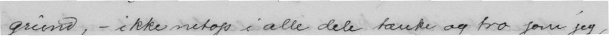grund, - ikke netop i alle dele tænke og tro som jeg,
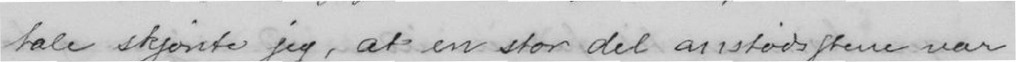tale skjønte jeg, at en stor del anstødsstene var
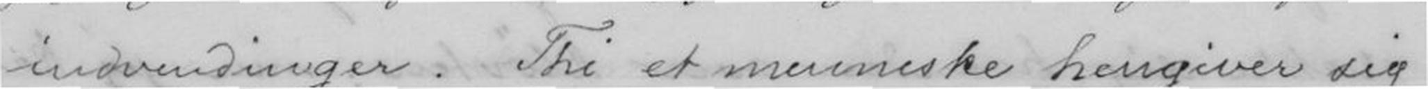indvendinger. Thi et menneske hengiver sig
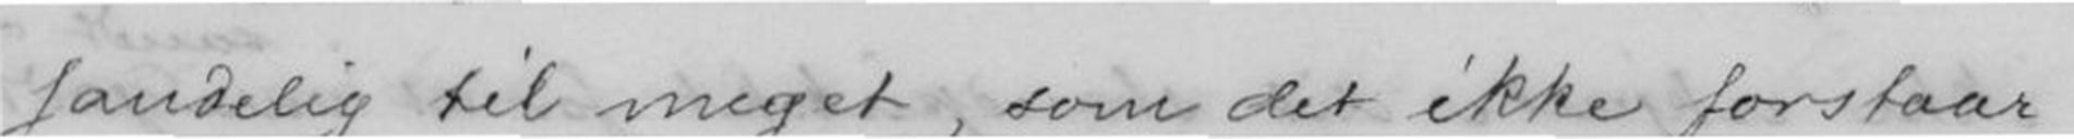sandelig til meget, som det ikke forstaar
